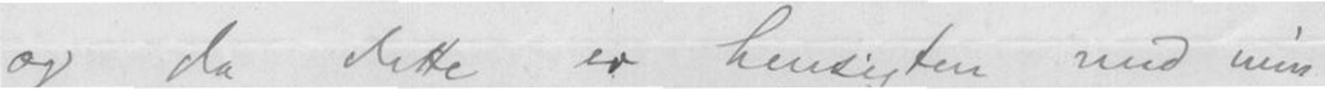og da dette er hensigten med min
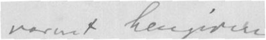varmt hengivne
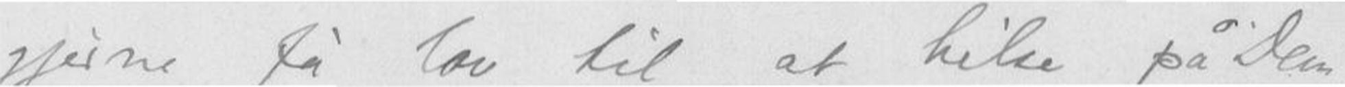gjerne få lov til at hilse på Dem,
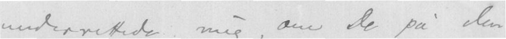underrettede mig, om De på den
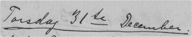Torsdag 31te December.
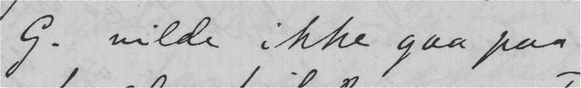G. vilde ikke gaa paa
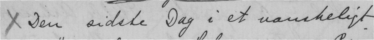x Den sidste Dag i et vanskeligt
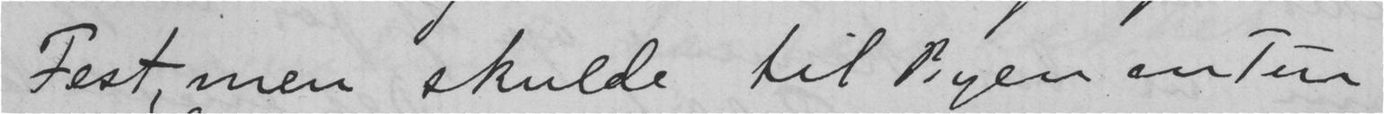Fest, men skulde til Byen en tur
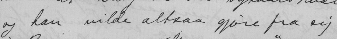og han vilde altsaa gjøre fra sig
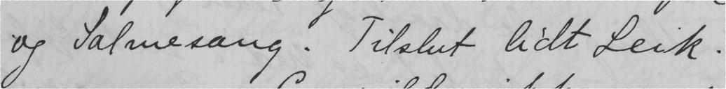og Salmesang. Tilslut lidt Leik.
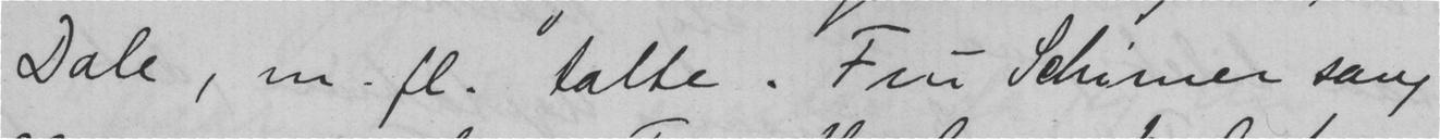Dale, m. fl. talte. Fru Schimer sang
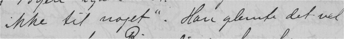ikke til noget". Han glemte det vel
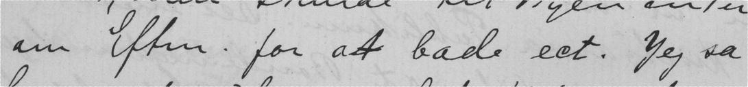om Eften. for at bade ect. Jeg sa
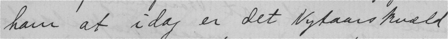ham at idag er det Nytaarskvæld
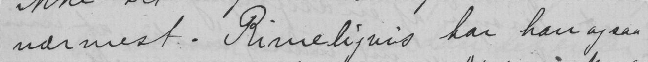nærmest. Rimeligvis har han ogsaa
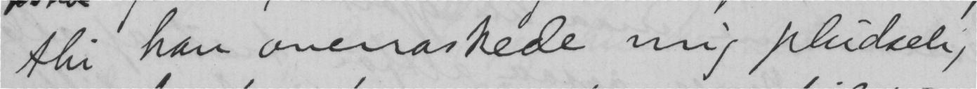thi han overraskede mig pludselig
